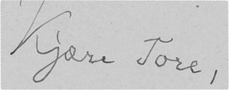Kjære Tore,
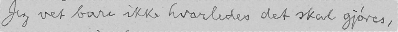Jeg vet bare ikke hvorledes det skal gjøres,
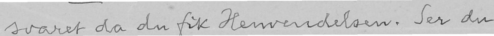svaret da du fik Henvendelsen. Ser du
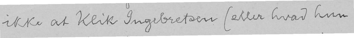ikke at Klik Ingebretsen (eller hvad hun
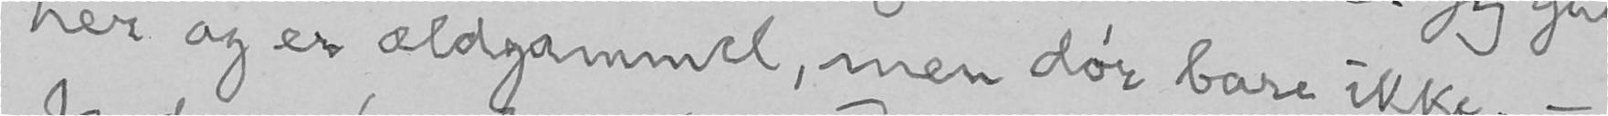her og er ældgammel, men dør bare ikke. -
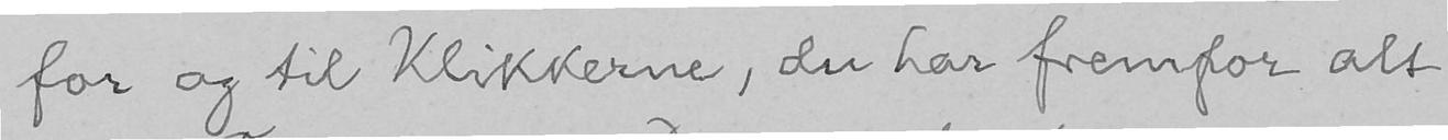for og til Klikkerne, du har fremfor alt
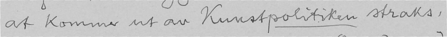at komme ut av Kunstpolitiken straks,
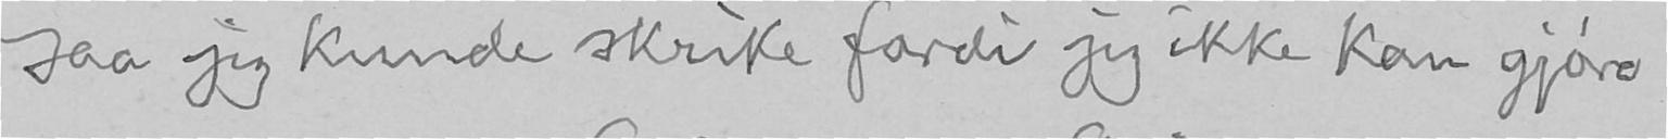saa jeg kunde skrike fordi jeg ikke kan gjøre
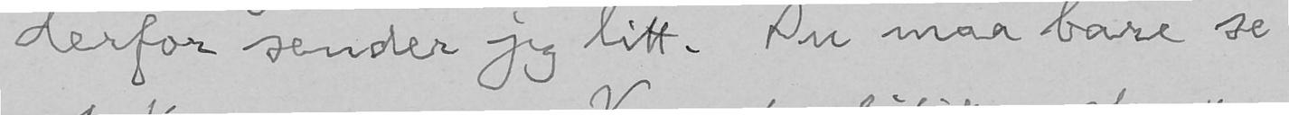derfor sender jeg litt. Du maa bare se
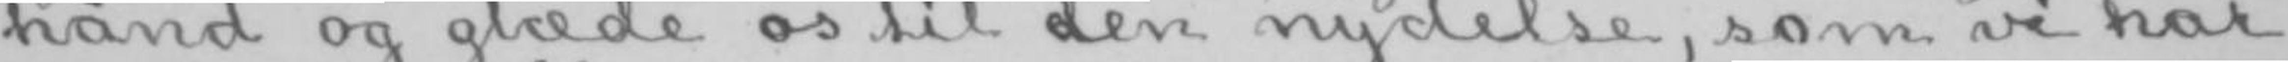hånd og glæde os til den nydelse, som vi har
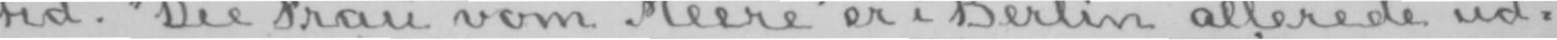tid. «Die Frau vom Meere» er i Berlin allerede ud-
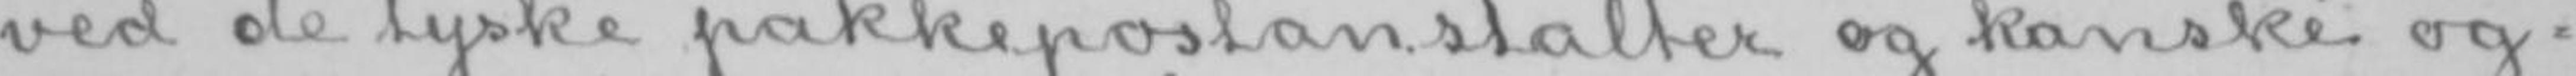ved de tyske pakkepostanstalter og kanské og-
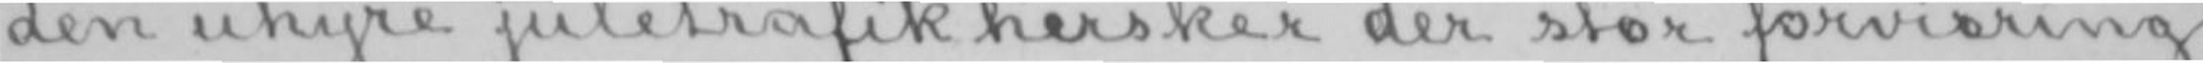den uhyre juletrafik hersker der stor forvisrring
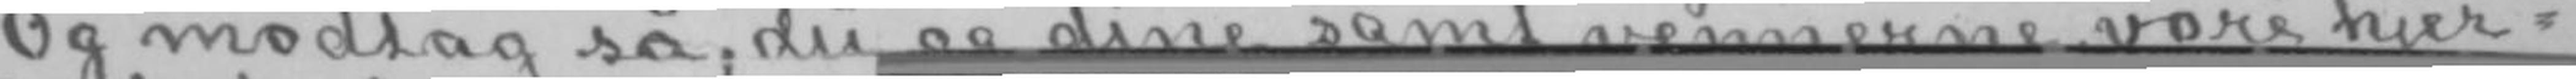Og modtag så, du og dine samt vennerne vore hjer-
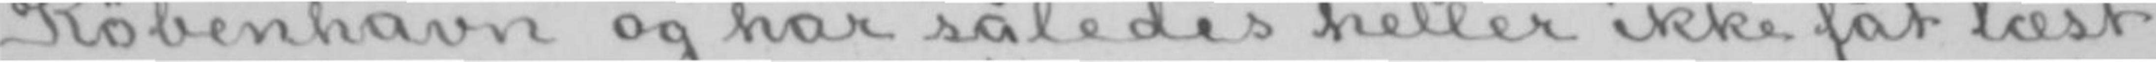København og har således heller ikke fåt læst
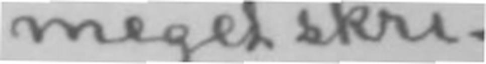meget skri-
